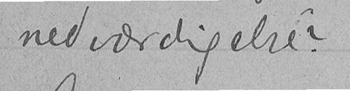nedværdigelse?
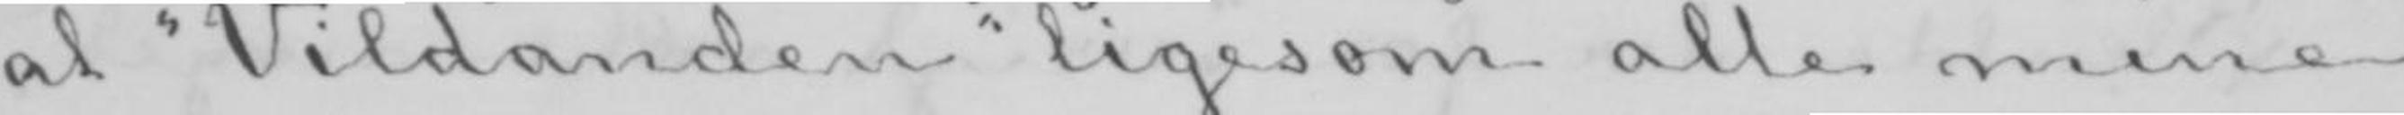at «Vildanden» ligesom alle mine
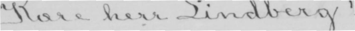Kære herr Lindberg!
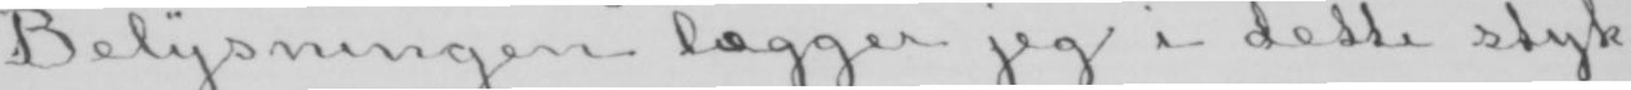Belysningen lægger jeg i dette styk-
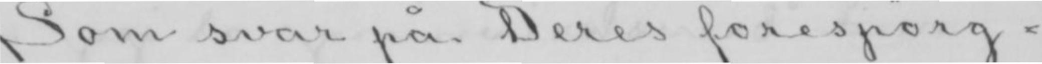Som svar på Deres forespørg-
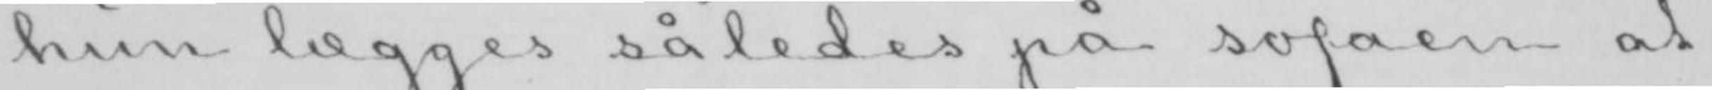hun lægges således på sofaen at
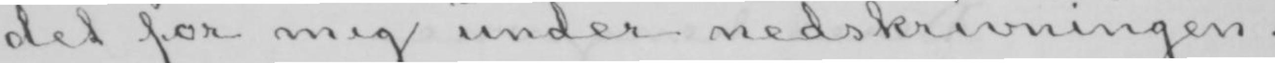det for mig under nedskrivningen.
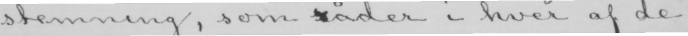stemning, som råder i hver af de
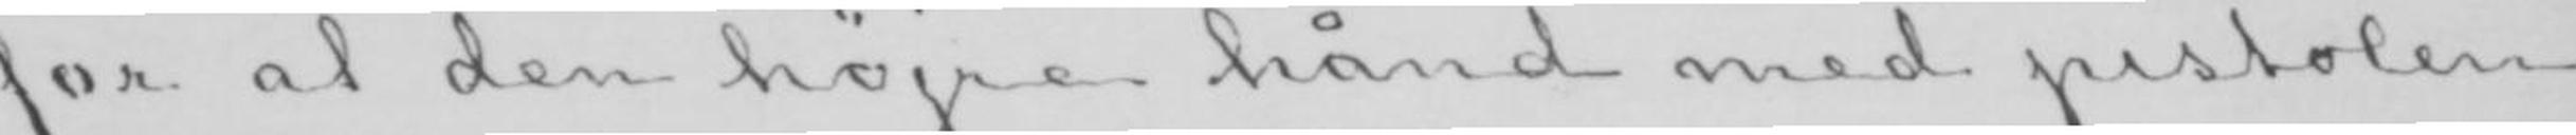for at den højre hånd med pistolen
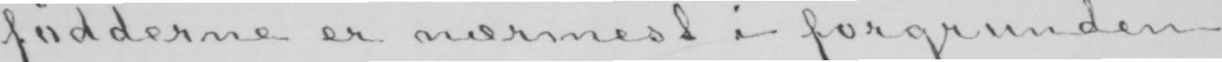fødderne er nærmest i forgrunden,
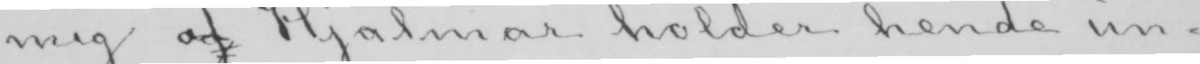mig ogat Hjalmar holder hende un-
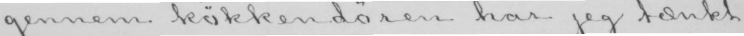gennem køkkendøren har jeg tænkt
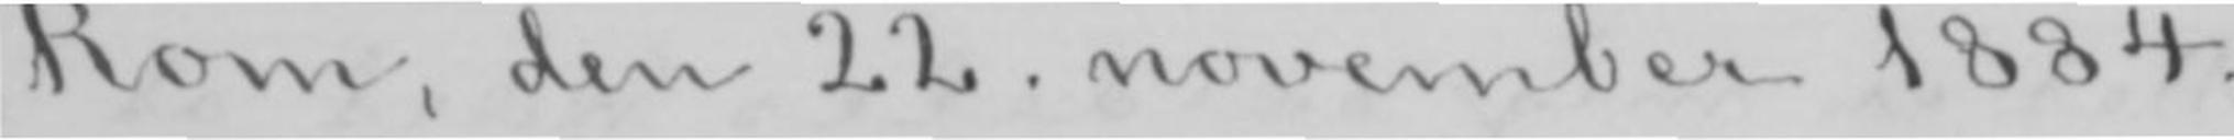Rom, den 22. november 1884.
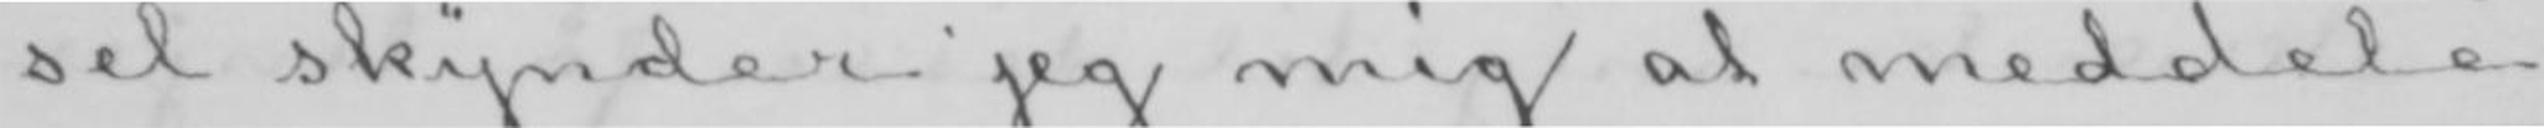sel skynder jeg mig at meddele
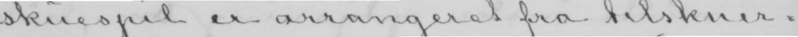skuespil er arrangeret fra tilskuer-
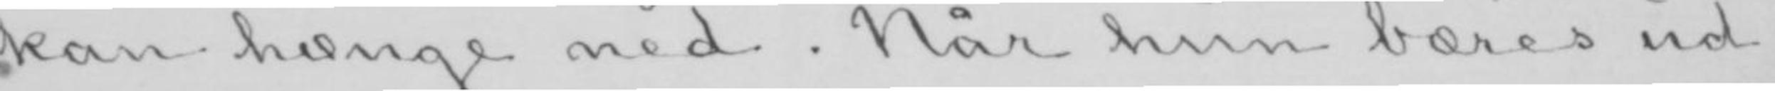kan hænge ned. Når hun bæres ud
